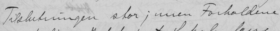Tilslutningen stor; men Forholdene
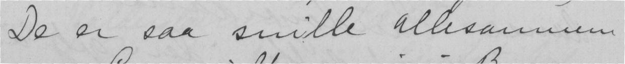De er saa snille allesammen
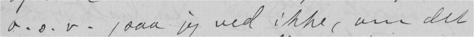o. s. v. (saa jeg ved ikke, om det
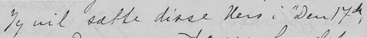Jeg vil sætte disse Vers i "Den 17de
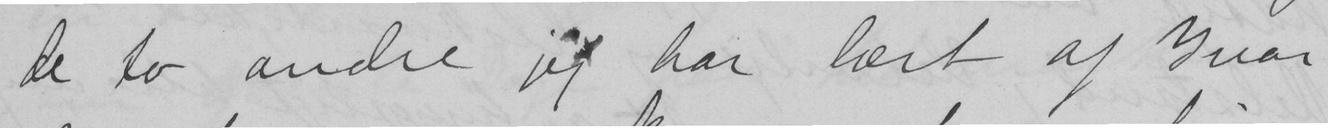de to andre jeg har lært af Ivar
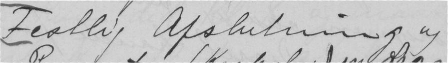Festlig Afslutning og
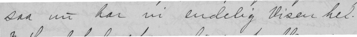saa nu har vi endelig Visen hel.
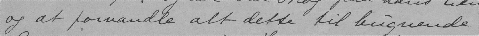og at forvandle alt dette til bugnende
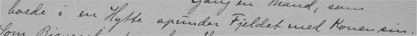boede i en Hytte opunder Fjeldet med Konen sin.
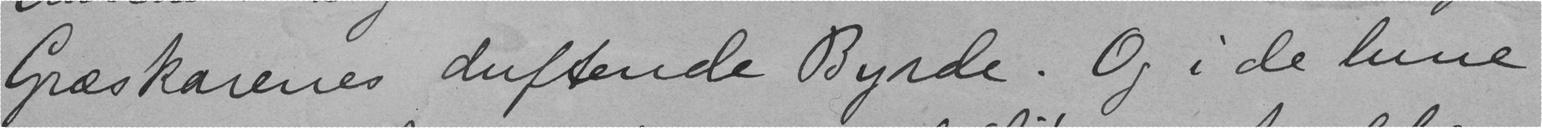Græskarenes duftende Byrde. Op i de lune
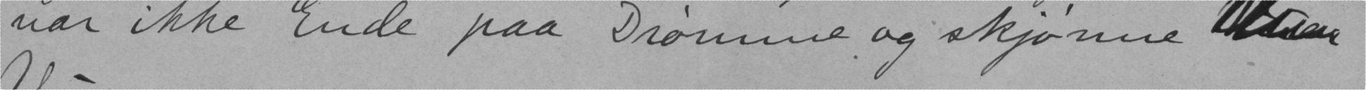var ikke Ende paa Drømme og skjønne
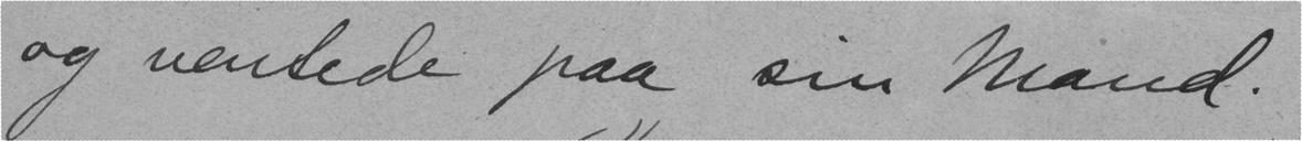og ventede paa sin Mand.
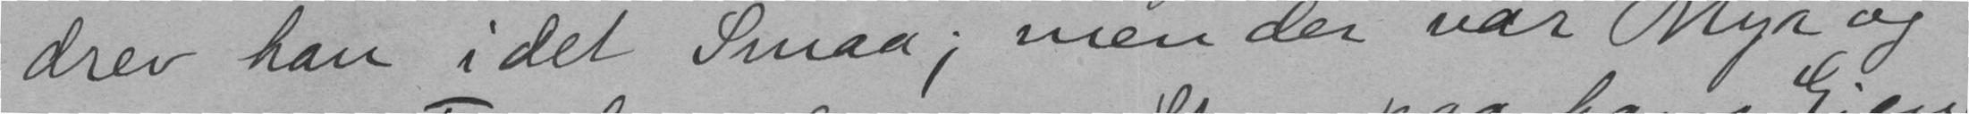drev han i det Smaa; men der var Myr og
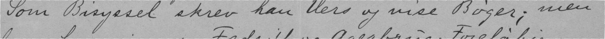Som Bisyssel skrev han Vers og vise Bøger; men
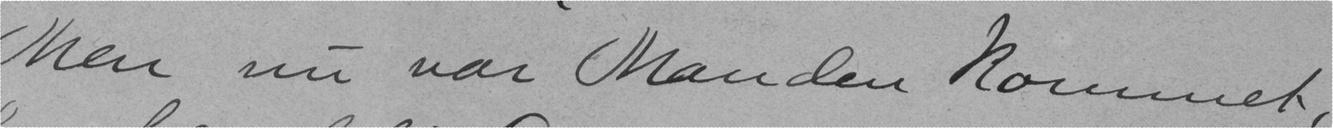Men nu var Manden kommet,
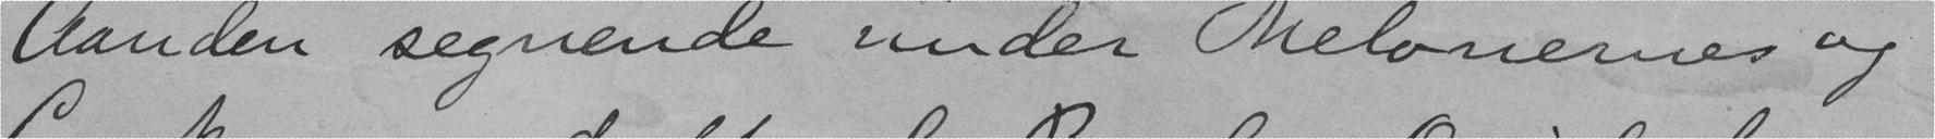Aanden segnende under Melonernes og
